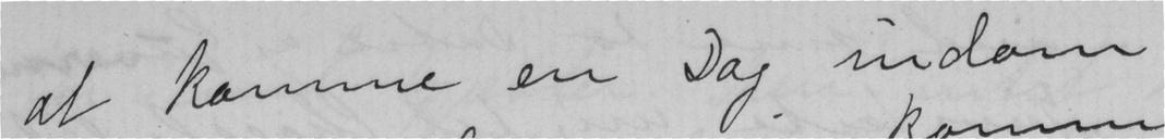at komme en Dag indom
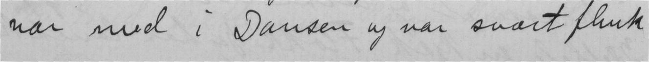var med i Dansen og var svært flink
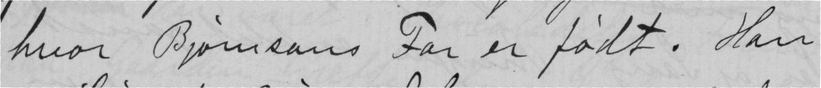hvor Bjørnsons Far er født. Han
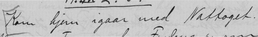Kom hjem igaar med Nattoget.
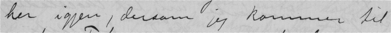her igjen, dersom jeg kommer til
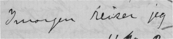I morgen reiser jeg
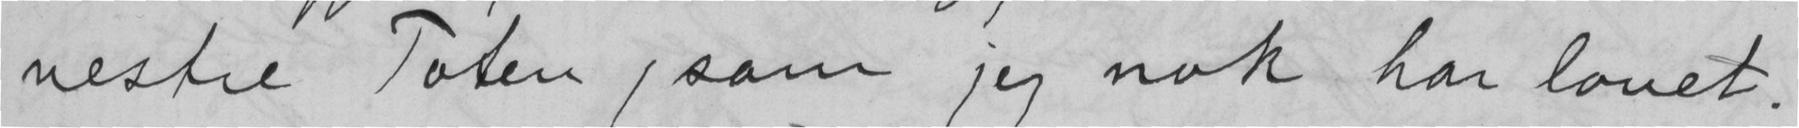vestre Toten, som jeg nok har lovet.
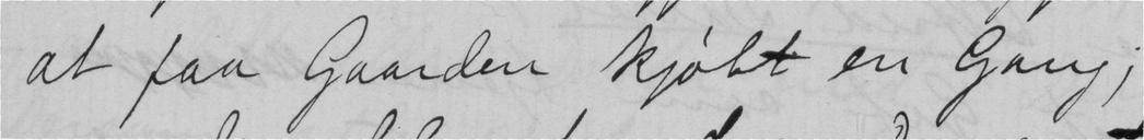at faa Gaarden kjøbt en Gang;
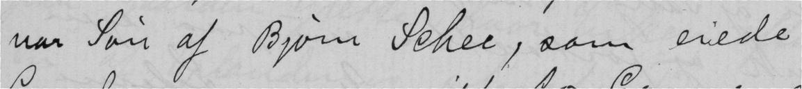var Søn af Bjørn Schee, som eiede
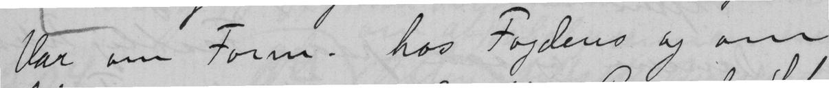Var om Form. hos Fogdens og om
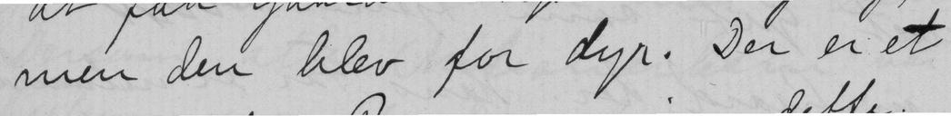men den blev for dyr. Der er et
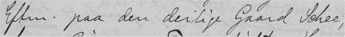Eftm. paa den deilige gaard Schee,
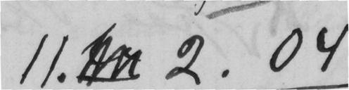11. 2.04
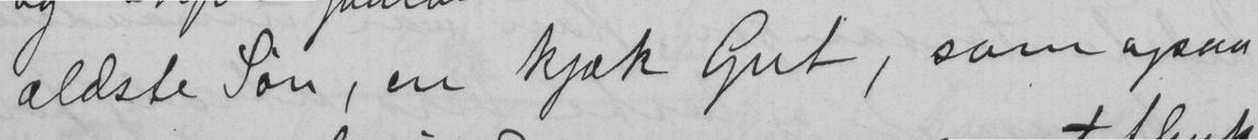ældste Søn, en kjæk Gut, som ogsaa
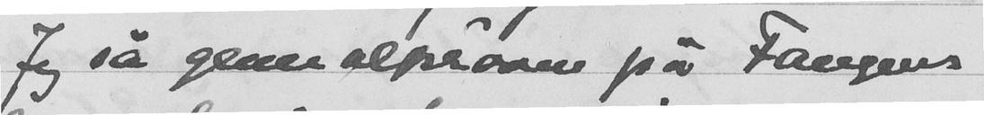Jeg så generalprøven på Fangens
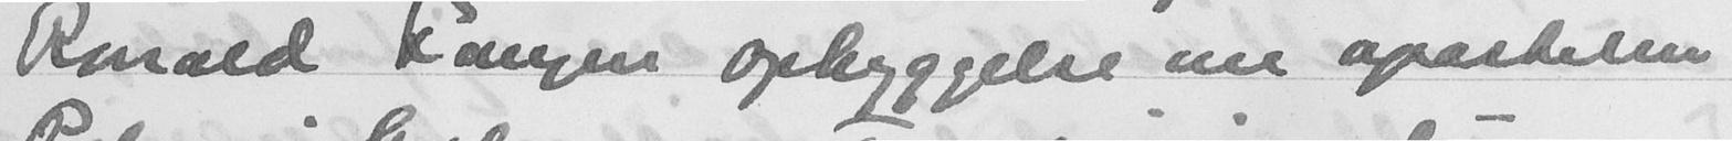Ronald Fangen opbyggelse om apostelen
